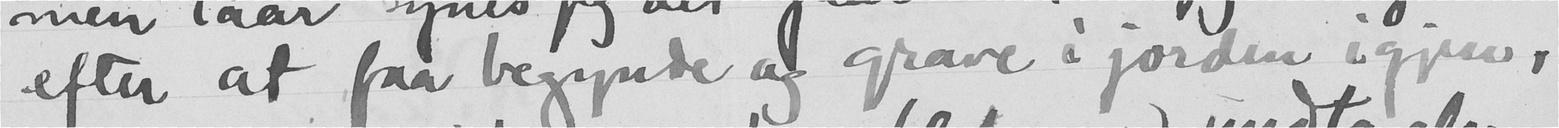efter at faa begynde og grave i jorden igjen,
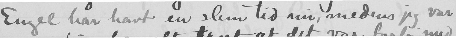Engel har havt en slem tid nu, medens jeg var
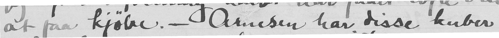at faa kjøbe. - Arnesen har disse kuber
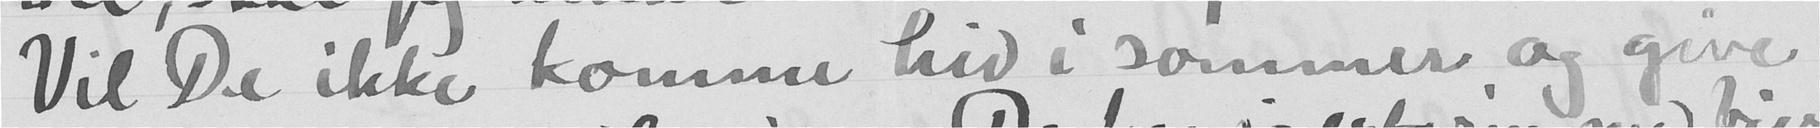Vil De ikke komme hid i sommer og give
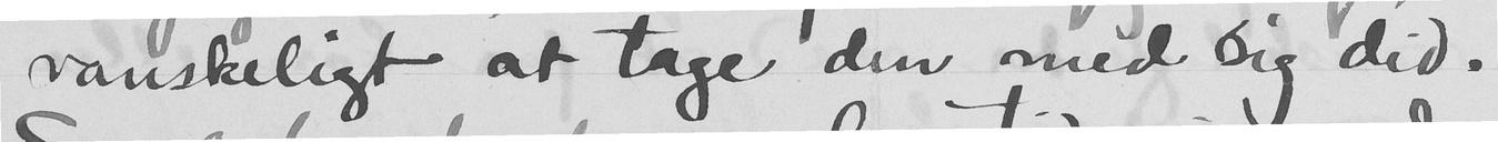vanskeligt at tage den med sig did.
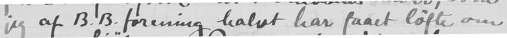jeg af B. B. forening halvt har faaet løfte om
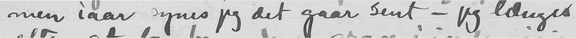men iaar synes jeg det gaar sent - jeg længes
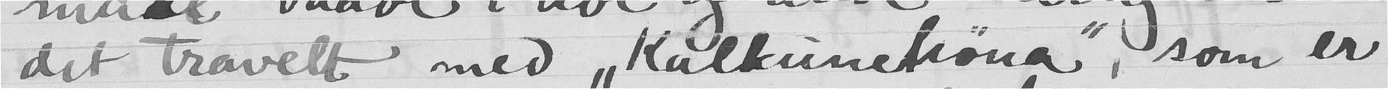det travelt med "Kalkunehøna", som er
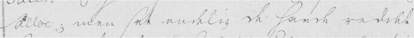Sallor; men set endelig de havde reddet
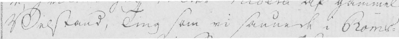Velstand, Ting som vi savnede i Roms-
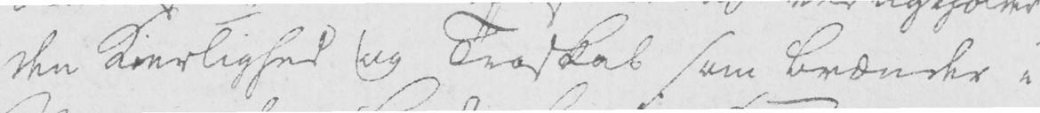den Kierlighed og Troskab som brænder i
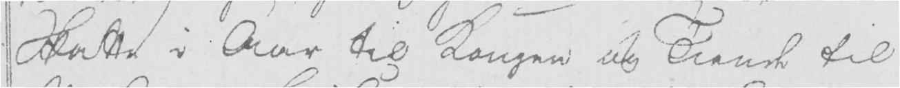Skatte i Aar til Kongen og Tiende til
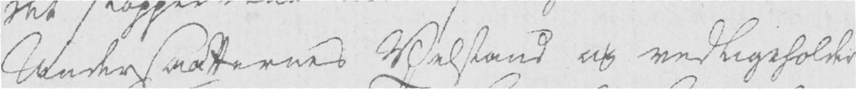Undersaatternes Velstand og vedligeholder
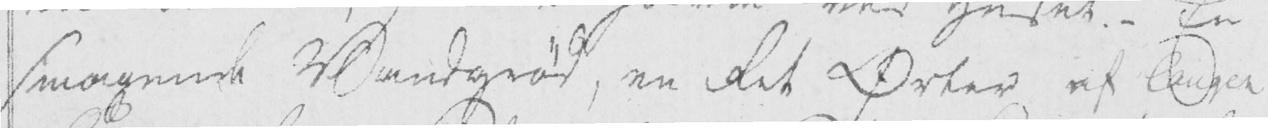smagende Vandgrød, en Ret Ørter af Laugen
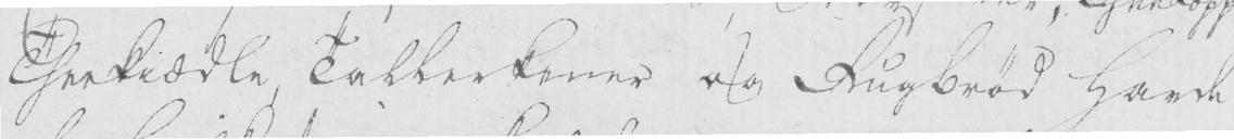Theekiædle, Tallerkener og Rugbrød havde
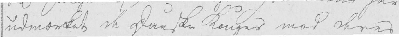udmærket de Danske Konger mod deres
